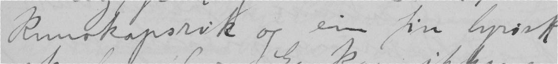kunnskapsrik og ein fin lyrisk
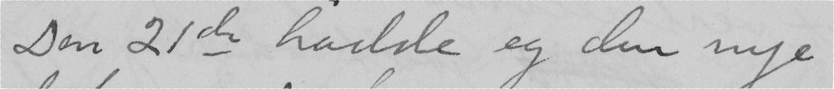Den 21de hadde eg den nye
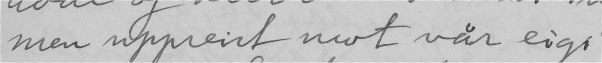men uppreist mot vår eigi
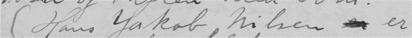Hans Jakob Nilsen  er
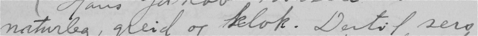naturleg, greid og klok. Dertil sers
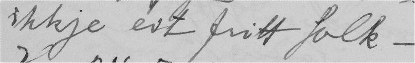ikkje eit fritt folk –
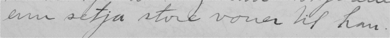enn setja store voner til han.
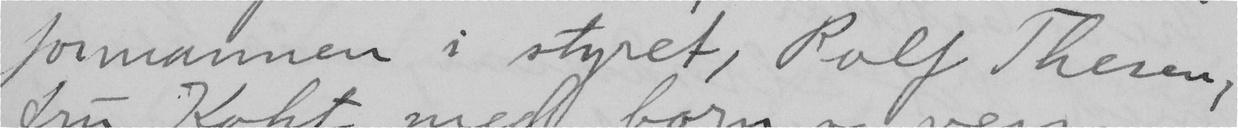formannen i styret, Rolf Thesen,
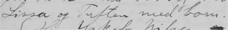Lissa og Tuften med born.
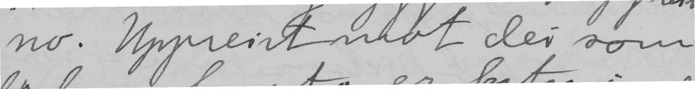no. Uppreist mot dei som
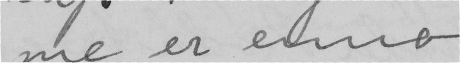me er enno
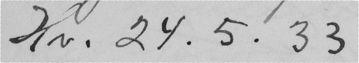Hv. 24.5.33
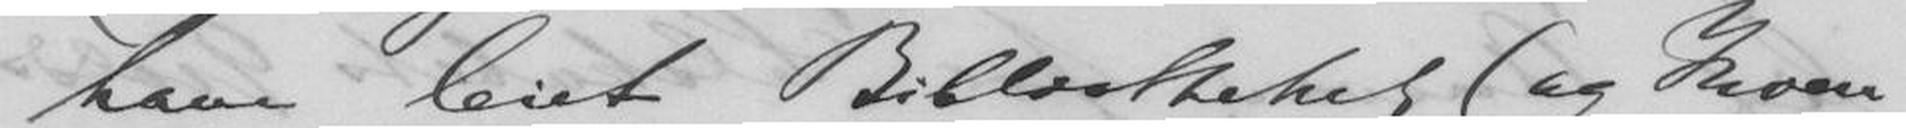have leiet Bibliothelet (og Inven-
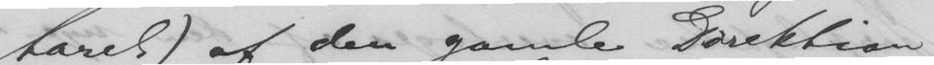taret) af den gamle Direktion
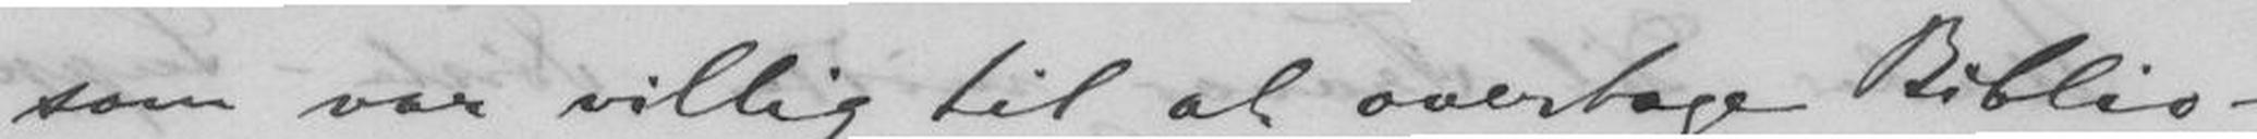som var villig til at overtage Biblio-
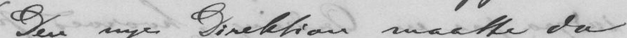(Den nye Direktion maatte da
